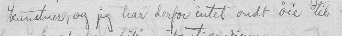kunstner, og jeg har derfor intet ondt øie til
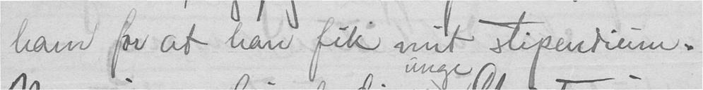ham for at han fik mit stipendium.
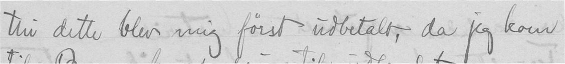thi dette blev mig først udbetalt, - da jeg kom
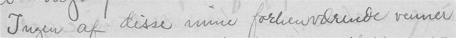Ingen af disse mine forhenværende venner
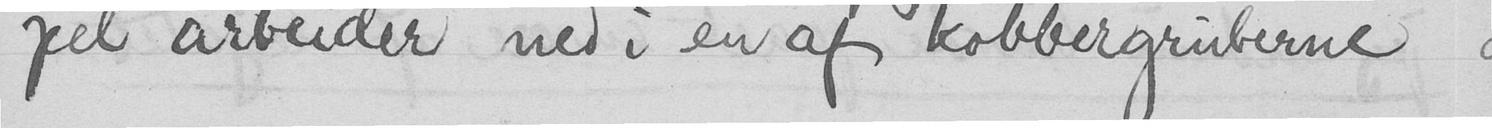pel arbeider ned i en af kobbergruberne
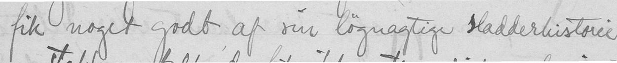fik noget godt af sin løgnagtige sladderhistorie
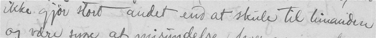ikke gjør stort andet ens at skule til hinanden
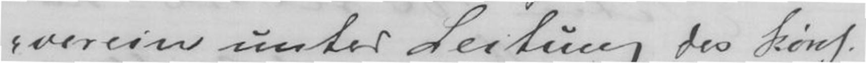verein unter Leitung des köns.
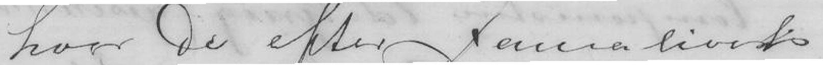hvor De efter Xanialivets
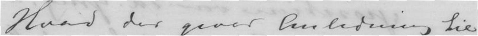Hvad der giver Anledning til
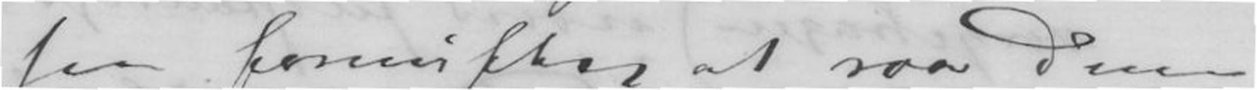som honniflag at roa Dem
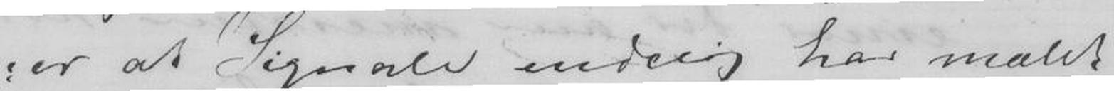er at Signale endelig har malet
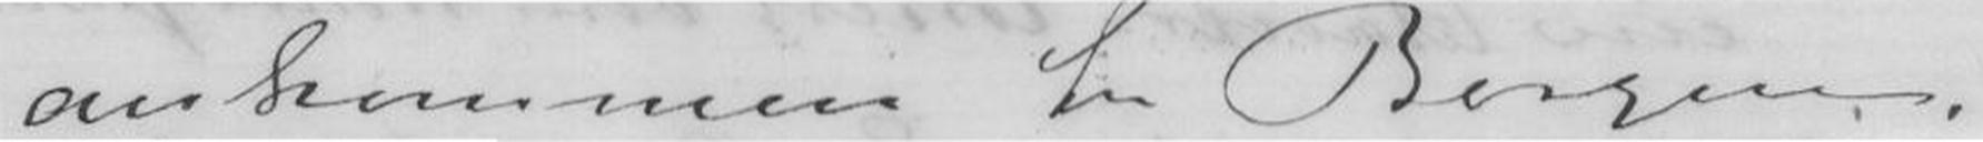ankommen til Bergen
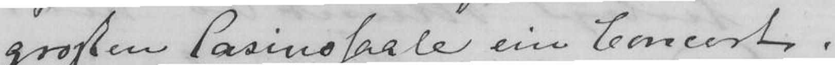großen Casinosaale ein Concert,
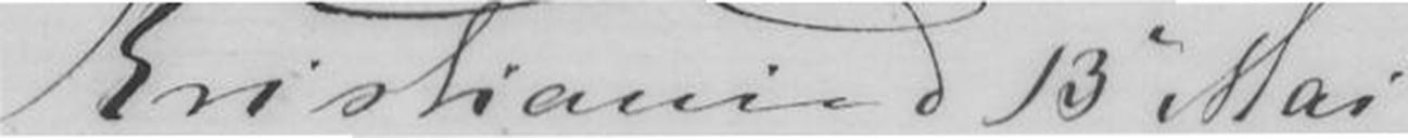Kristiania d 13 Mai
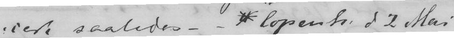certe saaledes - * "Copenh. d 2 Mai
